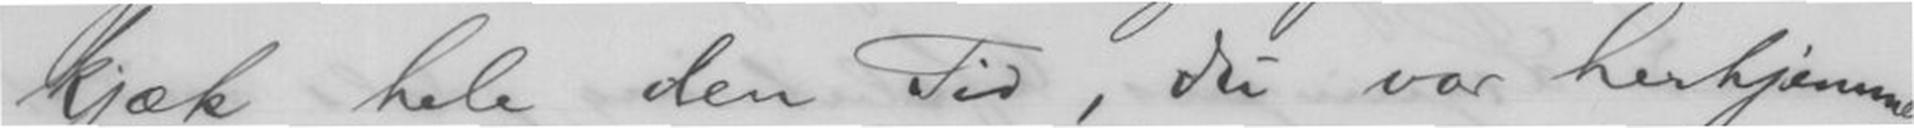kjæk hele den Tid, du var herhjemme.
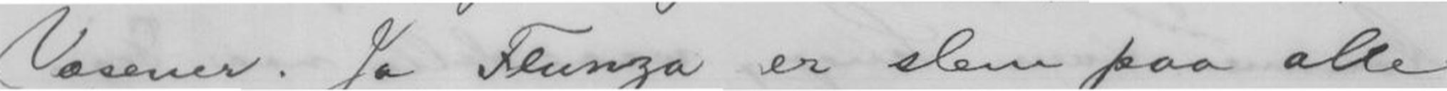Væsener. Ja Flunza er slem paa alle
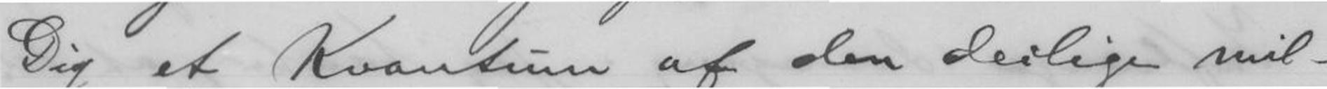Dig et Kvantum af den deilige mil-
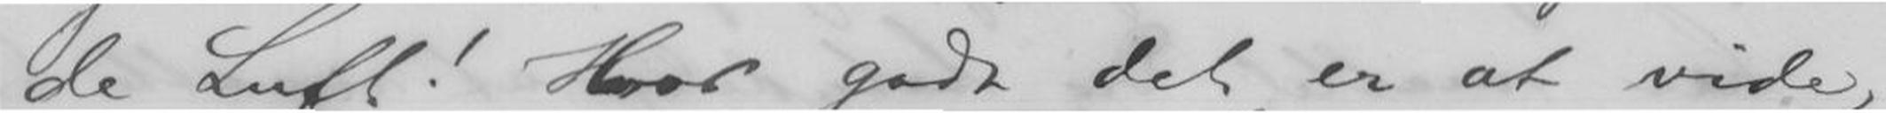de Luft! Hvor godt det er at vide,
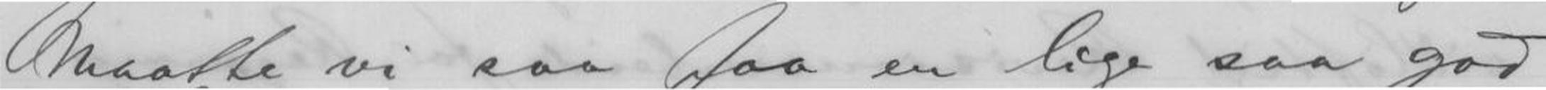Maatte vi saa faa en lige saa god
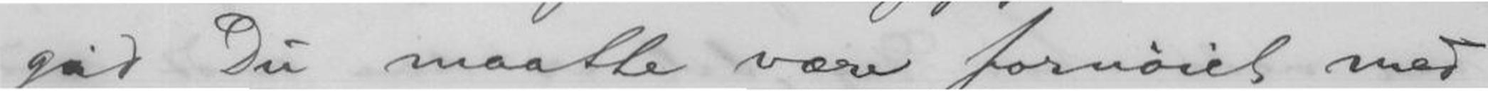gid Du maatte være fornøiet med
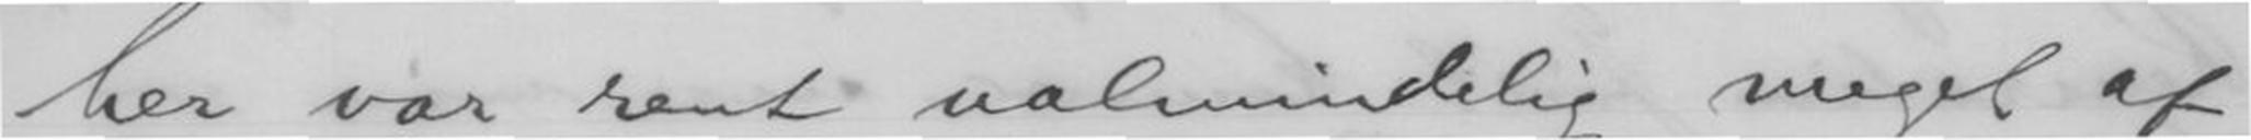her var rent ualmindelig meget af
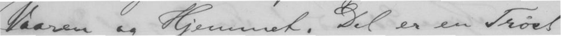Vaaren og Hjemmet. Det er en Trøst
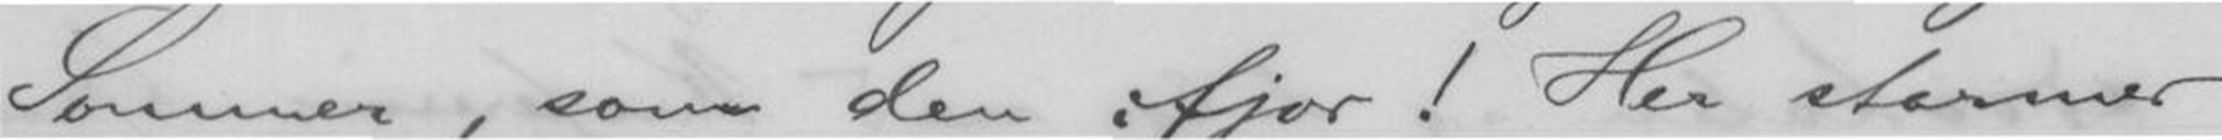Sommer, som den ifjor! Her stormer
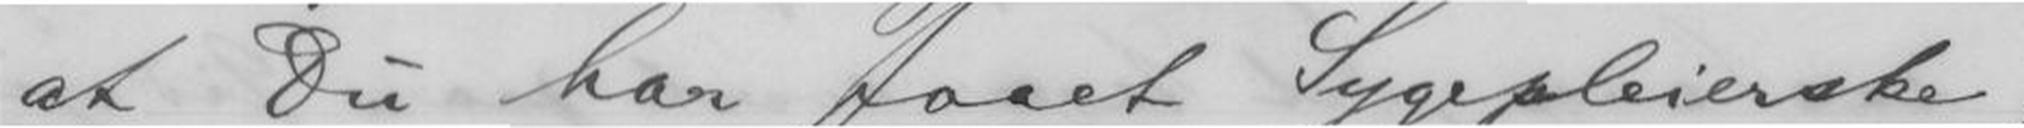at Du har faaet Sygepleierske,
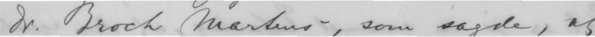Dr. Broch Martens, som sagde, at
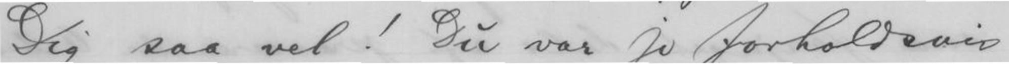Dig saa vel! Du var jo forholdsvis
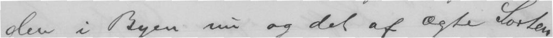den i Byen nu og det af ægte Sorten.
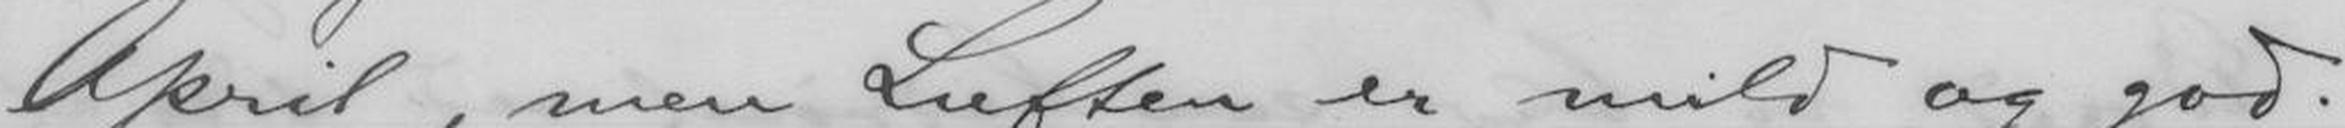April, men Luften er mild og god.
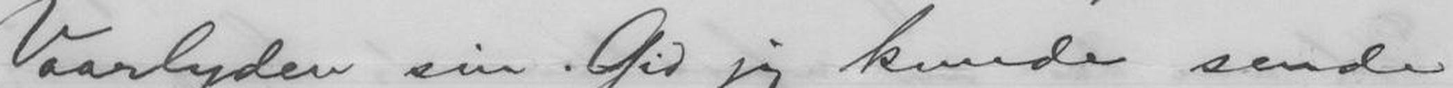Vaarlyden sin. Gid jeg kunde sende
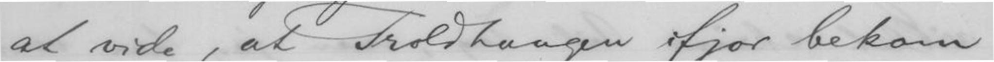at vide, at Troldhaugen ifjor bekom
